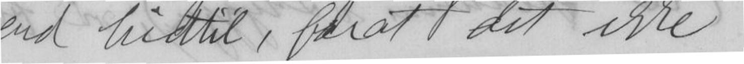end hidtil, forat det ikke
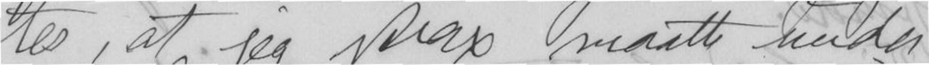tes, at jeg strax maatte under-
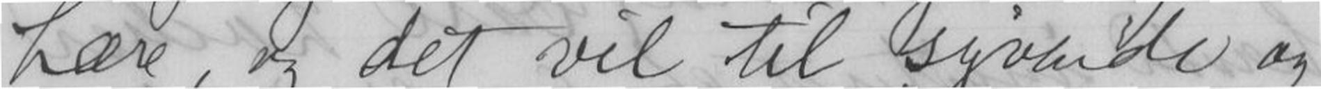lære, og det vil til syvende og
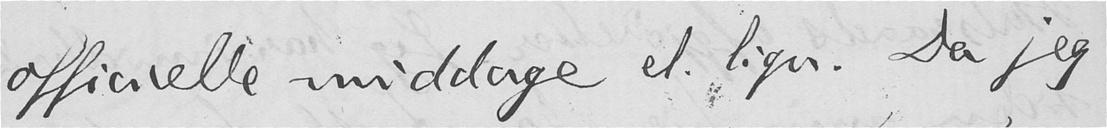officielle middage el. lign. Da jeg
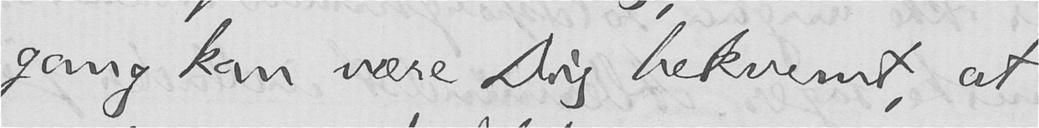gang kan være Dig bekvemt, at
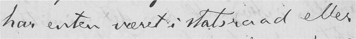har enten været i statsraad eller
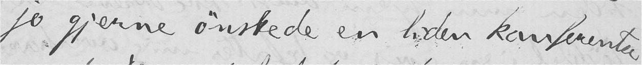jo gjerne ønskede en liden konferentse
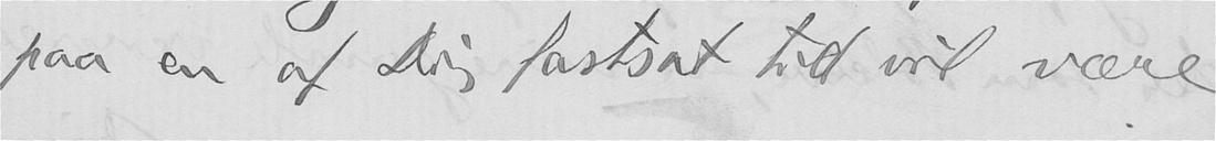paa en af Dig fastsat tid vil være
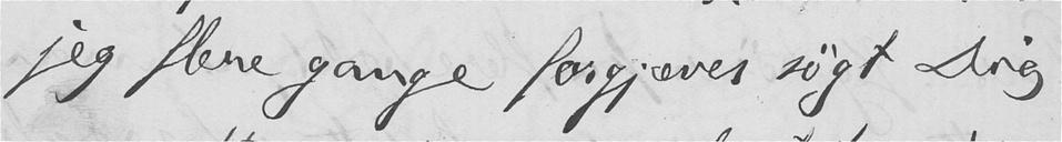jeg flere gange forgjæves søgt Dig
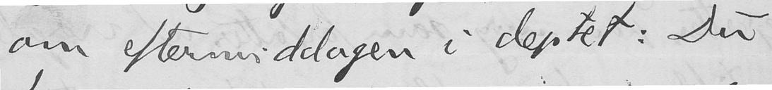om eftermiddagen i deptet: Du
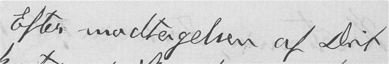Efter modtagelsen af Dit
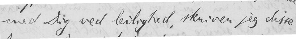med Dig ved leilighed, skriver jeg disse
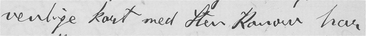venlige kort med Sten Konow har
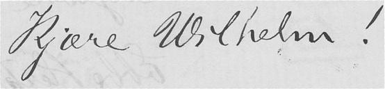Kjære Wilhelm!
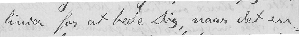linier for at bede Dig, naar det en-
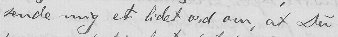sende mig et lidet ord om, at Du
